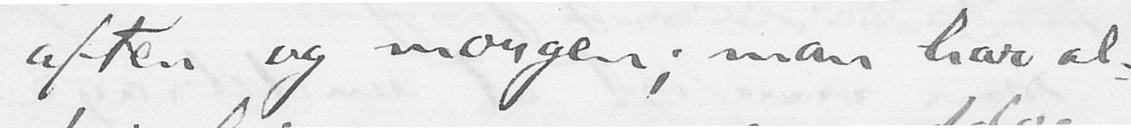aften og morgen; man har al-
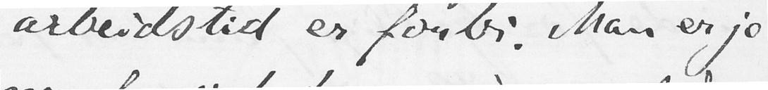arbeidstid er forbi. Man er jo
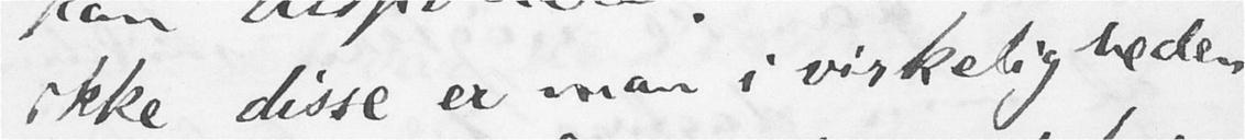ikke disse er man i virkeligheden
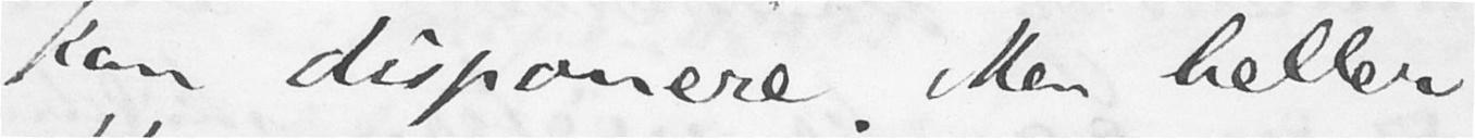kan disponere. Men heller
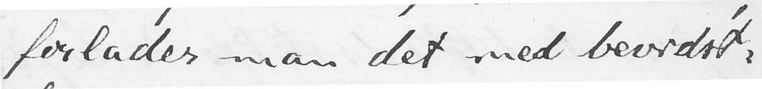forlader man det med bevidst-
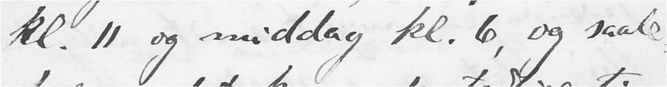kl. 11 og middag kl. 6, og saale-
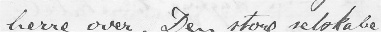herre over. Den store selskabe-
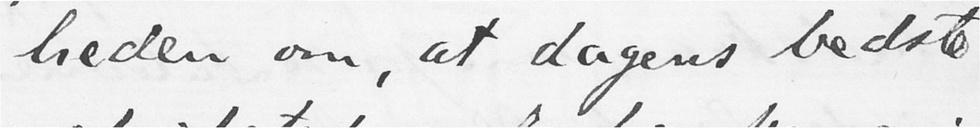heden om, at dagens bedste
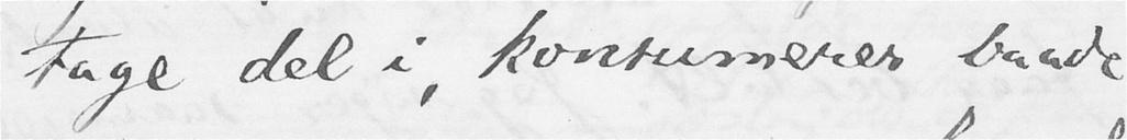tage del i, konsumerer baade
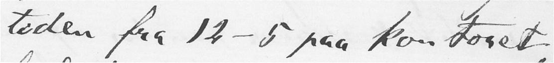tiden fra 12-5 paa kontoret,
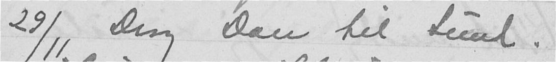29/11 Drog Don til Sund.
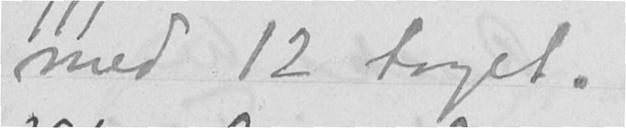med 12 toget.
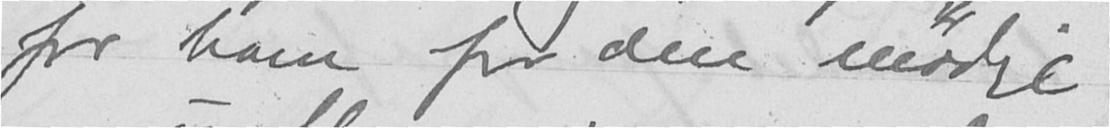for ham for den modige
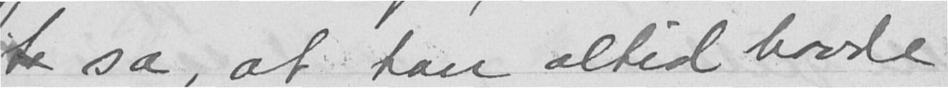te sa, at han altid havde
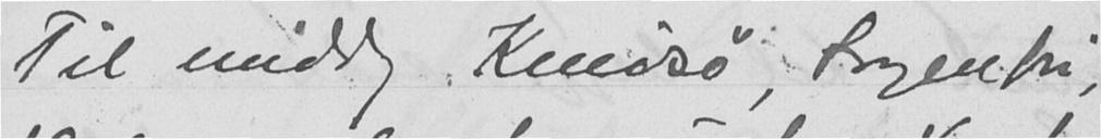Til middag Knivsø, Sorgenfri,
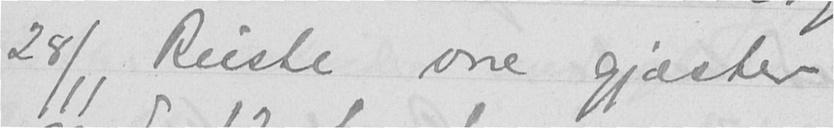28/11 Reiste vore gjæster
